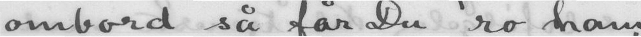ombord så får Du ro ham
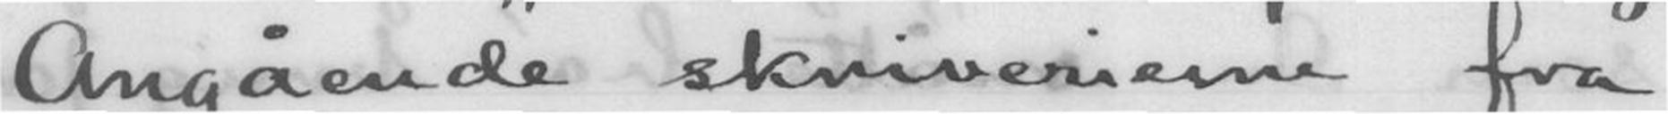Angående skriverierne fra
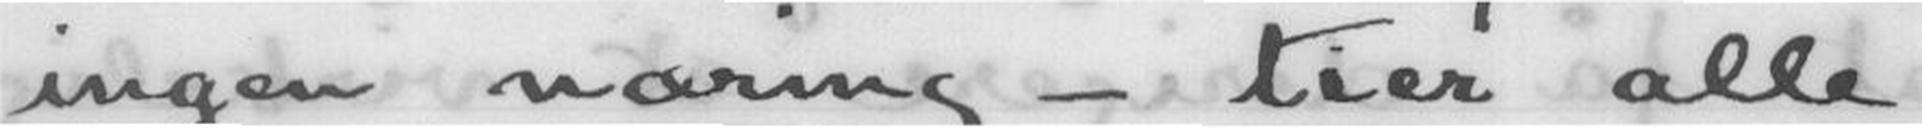ingen næring - tier alle
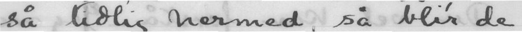så tidlig hermed, så bli'r de
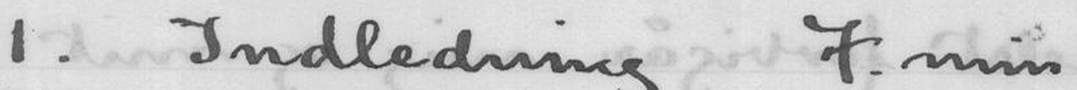1. Indledning7. min
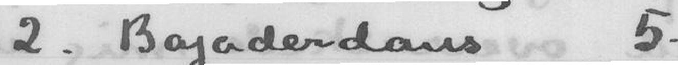2. Bajaderdans 5.
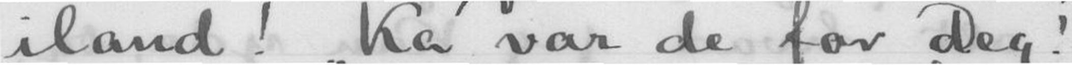iland! Ka' var de for Deg!
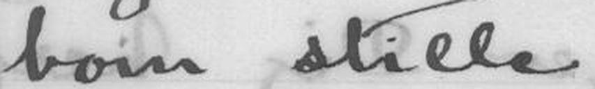bom stille.
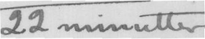22 minutter
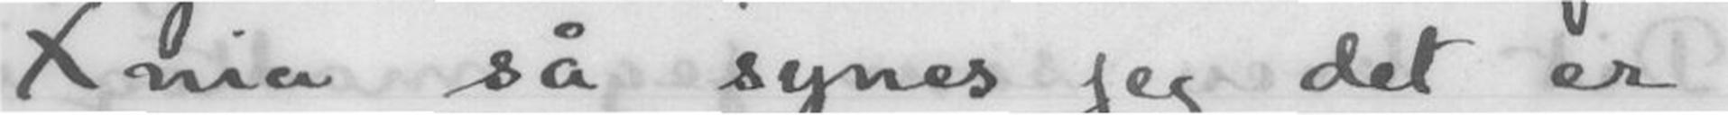Xania så synes jeg det er
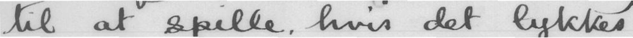til at spille, hvis det lykkes
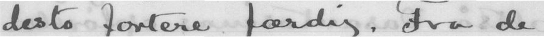desto fortere færdig. Fra de
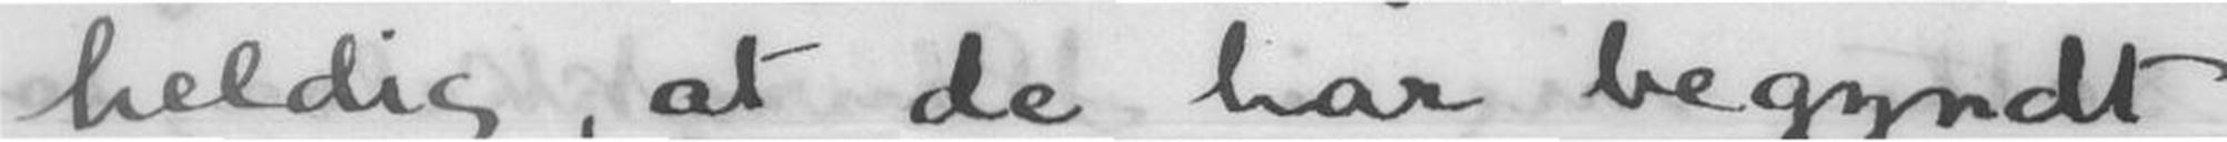heldig, at de har begyndt
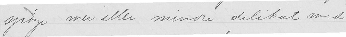spøge mer eller mindre delikat med
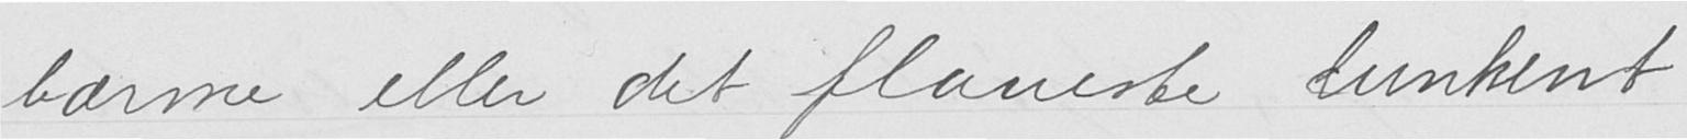barme eller det flaueste lunkent
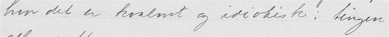hvor det er kvalmt og idiotisk: tingen
